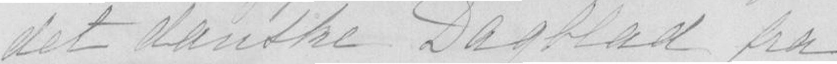det danske Dagblad fra
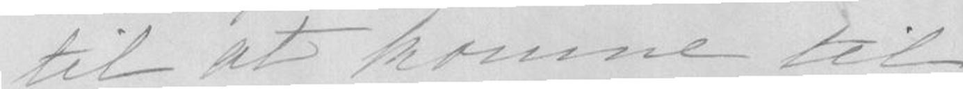til at komme til
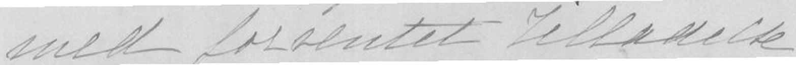med forventet Tilladelse
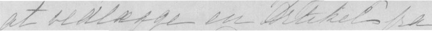at vedlægge en Artikel fra
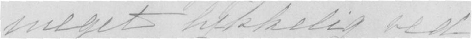meget lykkelig ved
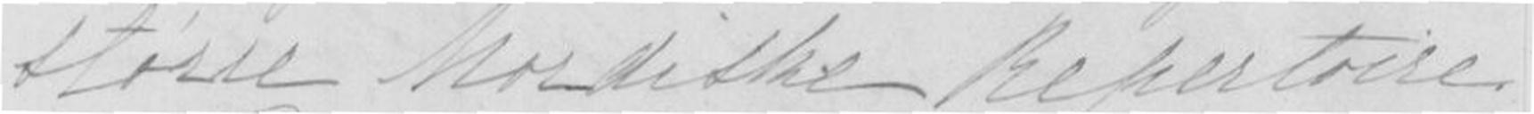større Nordiske Repertoire.
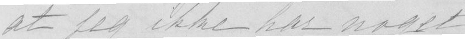at jeg ikke har noget
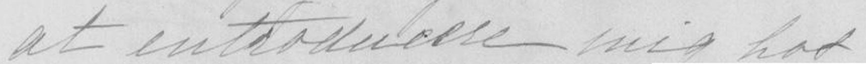at introducere mig hos
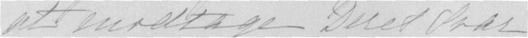at modtage Deres Svar
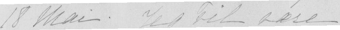18 Mai. Jeg vil være
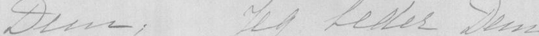Dem. Jeg beder Dem
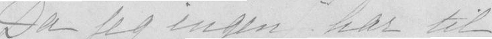Da jeg ingen har til
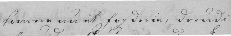som ere nu et Fogderie, derudi
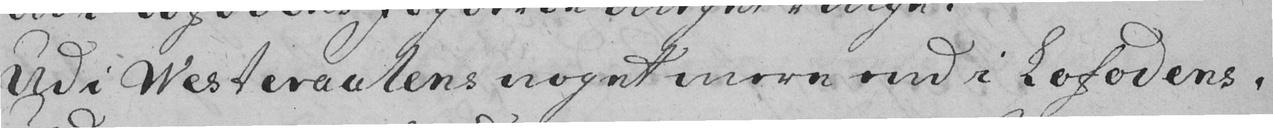Udi Westeraalens noget mere end i Lofodens.
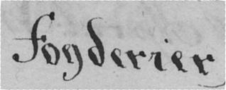Fogderier
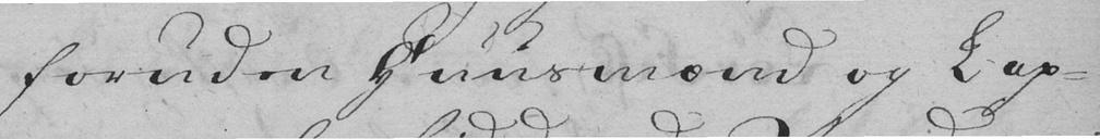foruden Huusmænd og Lap-
